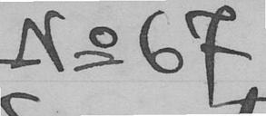No 67
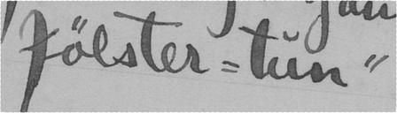Jølster-tun"
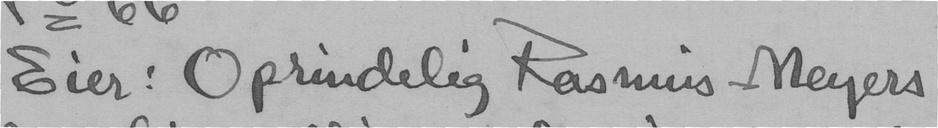Eier: Oprindelig Rasmus Meyers
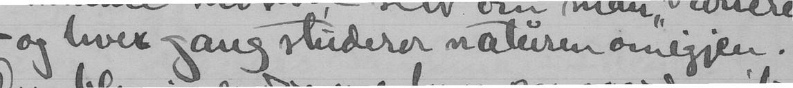og hver gang studerer naturen omigjen.
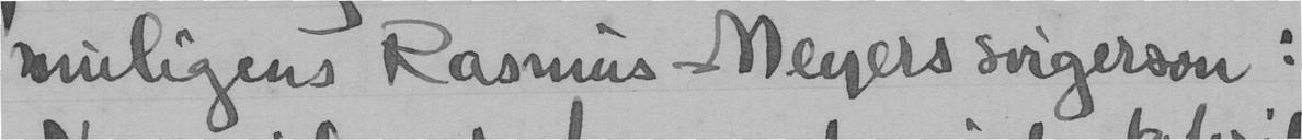muligens Rasmus Meyers svigersøn:
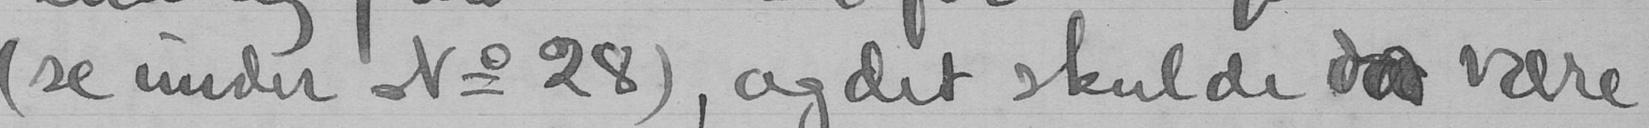(se under No 28), og det skulde da være
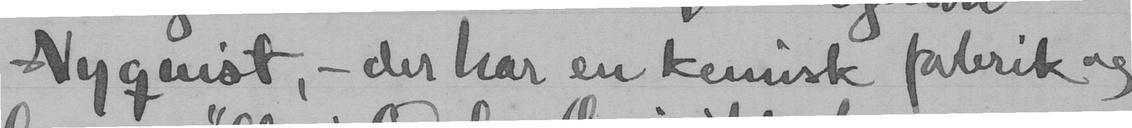Nyquist, - der har en kemisk fabrik og
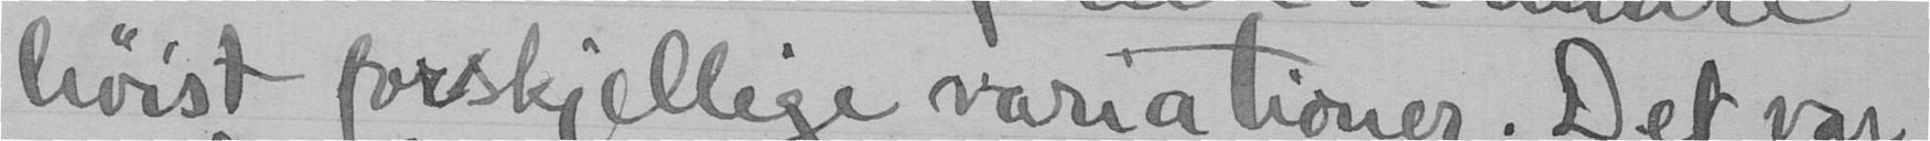høist forskjellige variationer. Det var
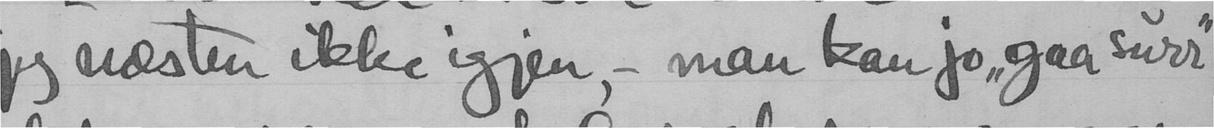jeg næsten ikke igjen - man kan jo "gaa surr"
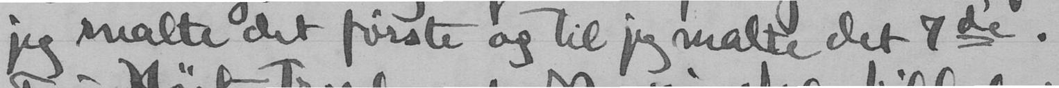jeg malte det første og til jeg malte det 7de.
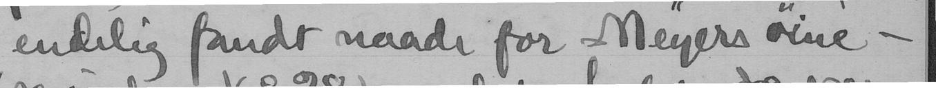endelig fandt naade for - Meyers øine -
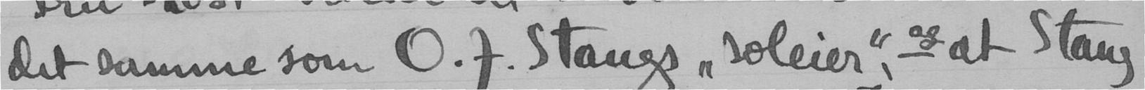det samme som O.J. Stangs "soleier" og at Stang
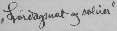"Lørdagsnat og soleier"
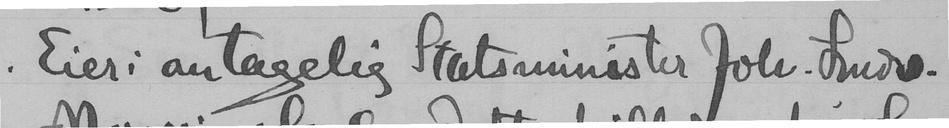Eier: antagelig Statsminister Joh. Ludv.
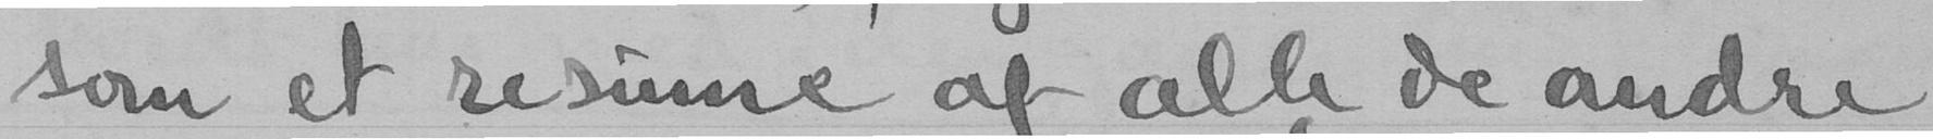som et resume af alle de andre
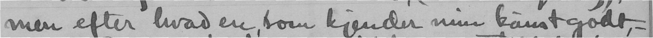men efter hvad en som kjender min kunst godt, -
